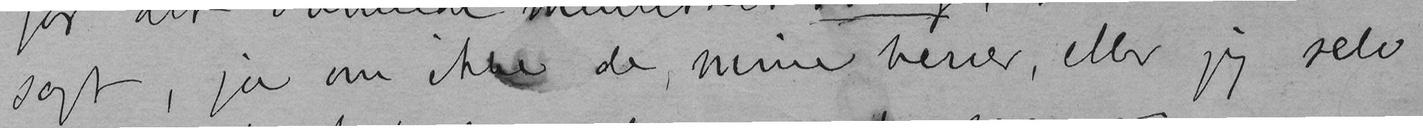sagt, ja om ikke de, mine herrer, eller jeg selv
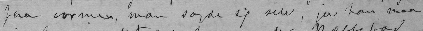paa varmen, man sagde sig selv, ja han maa
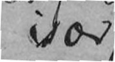især
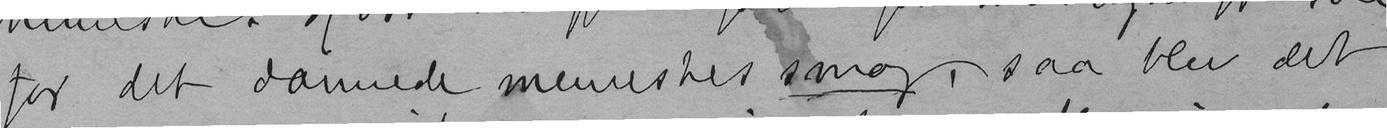for det dannede menneskes smag, saa blev det
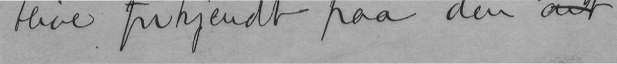blive frikjendt paa den art
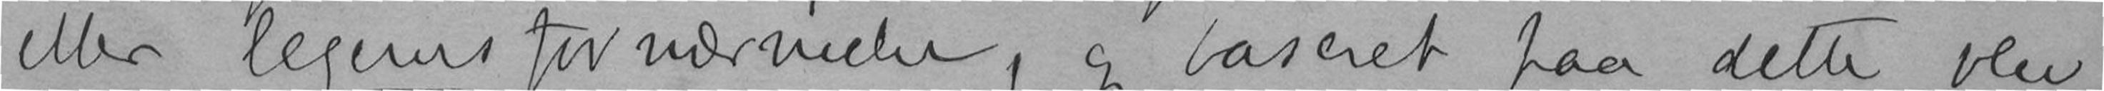eller legemsfornærmelse, og baseret paa dette blev
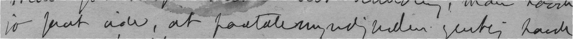jo faat vide, at paatalemyndigheden egentlig havde
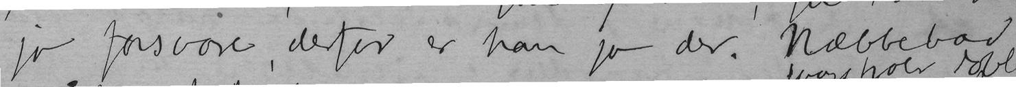jo forsvare, derfor er han jo der. Næbbebad
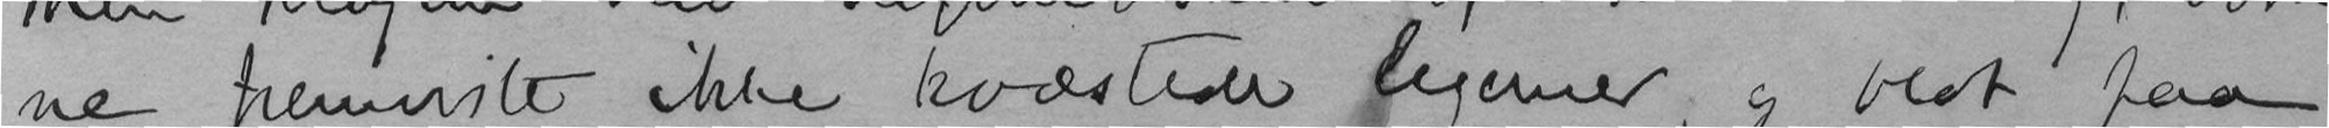ne fremviste ikke kvæstede legemer, og blot paa
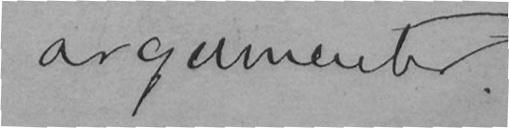argumenter.
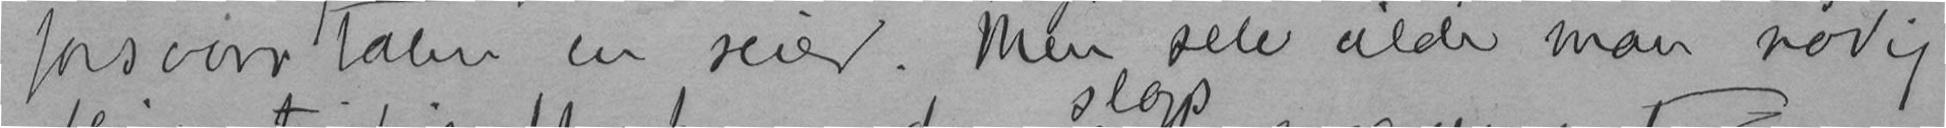forsvarstalen en seier. Men selv vilde man nødig
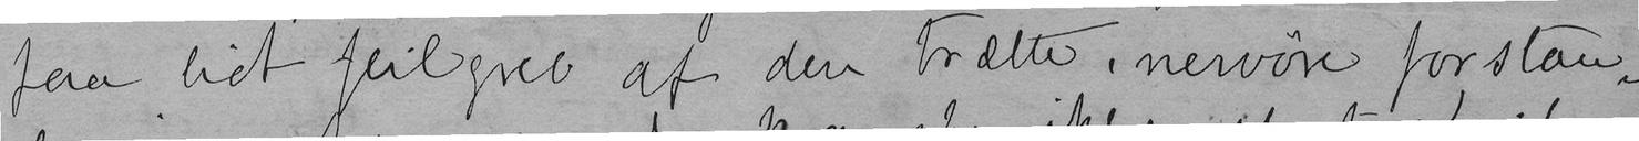paa lidt feilgreb af den trætte, nervøse forstan-
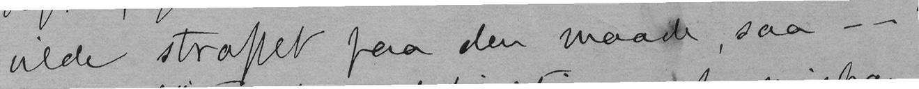vilde straffet paa den maade, saa - -
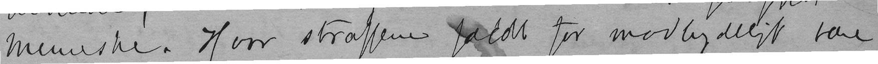Menneske. Hvor straffene faldt for modbydeligt bare
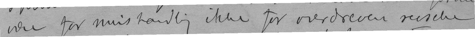være for mishandling ikke for overdreven revselse
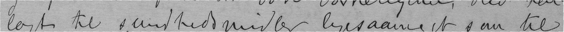lagt til sundhedsmidler ligesaameget som til
